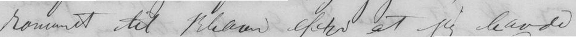kommet til Khavn efter at jeg havde
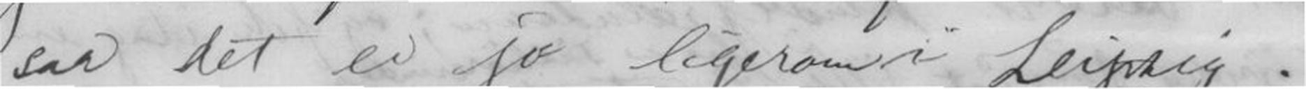saa der er jo ligesom i Leipzig.
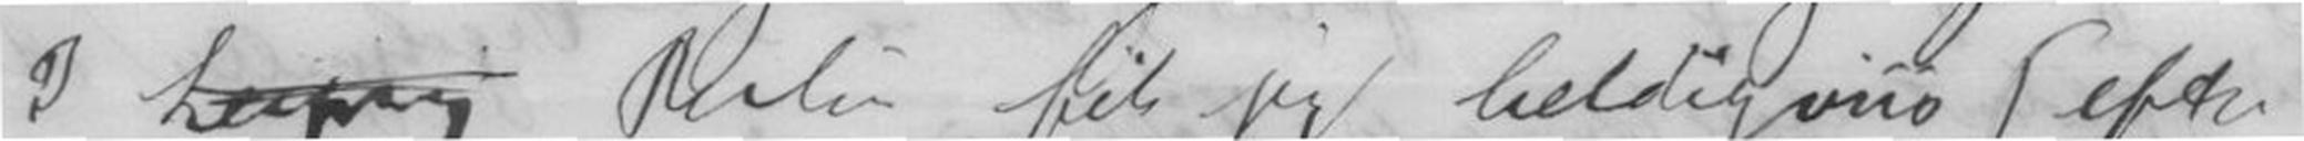I Leipzig Berlin fik jeg heldigvis (efter-
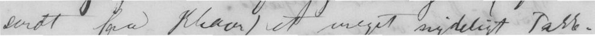sendt fra Khavn) et meget nydeligt Takke-
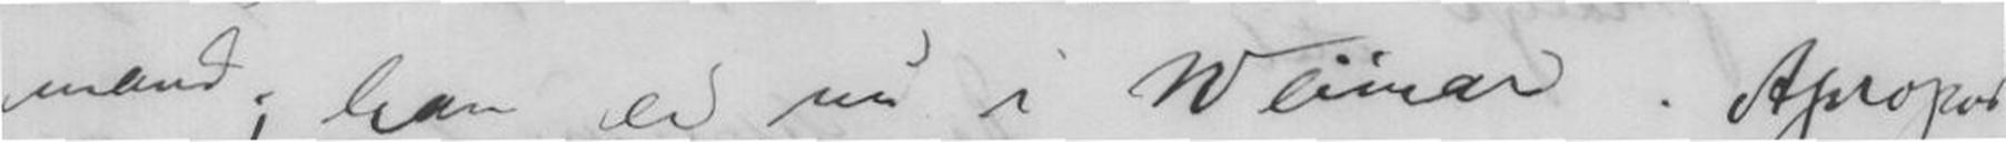mand; han er nu i Weimar. Apropos
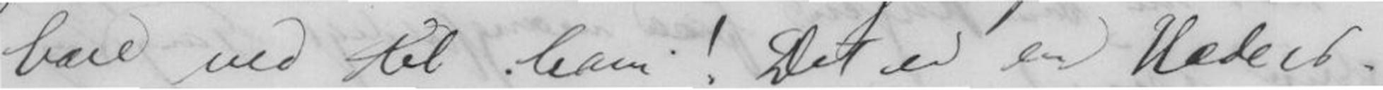bare ned til ham! Det er en Heders-
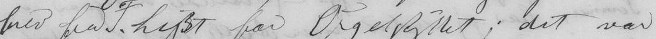brev fra F. Lizst for Orgelstykket; det var
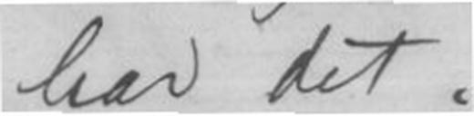har det.
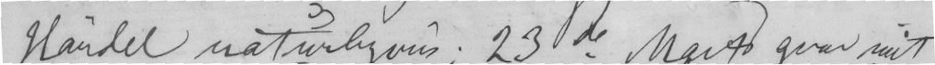Händel naturligvis; 23de Marts giver mit
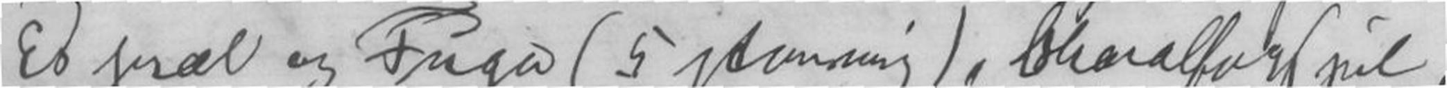Es præl og Fuger (5 stemmig), Choralforspil,
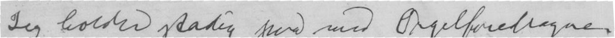Jeg holder stadig paa med Orgelforedragene
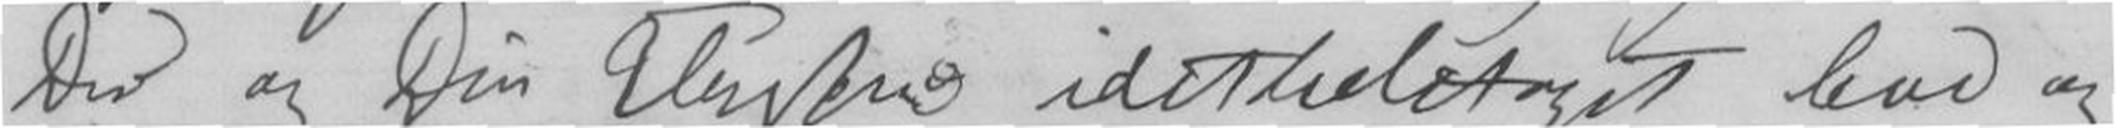Du og Din Hustru idet hele taget leve og
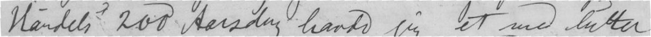Händels 200 Aarsdag havde jeg et med lutter
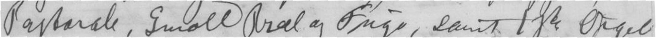Pastorale, Gmoll Præl og Fuge, samt 1ste Orgelcon-
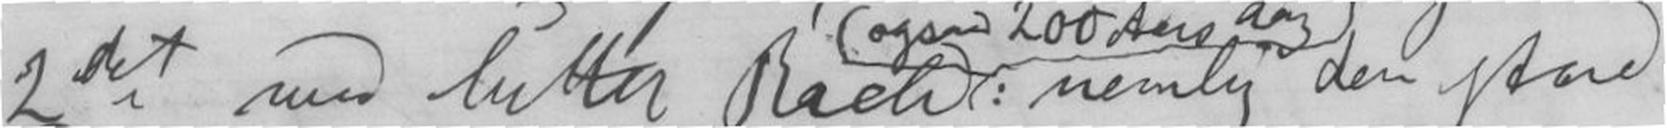2det med lutter Bach: nemlig den store
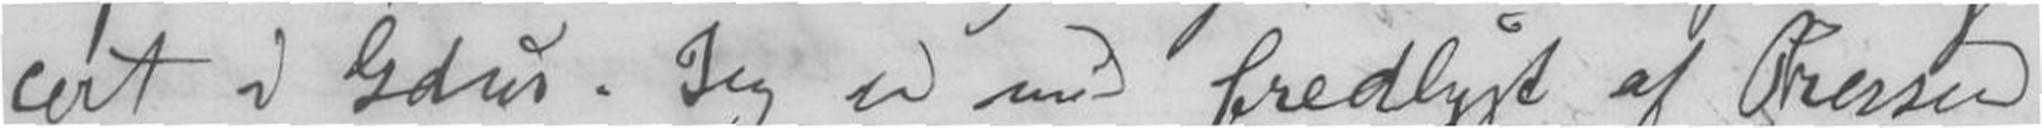cert i Gdur. Jeg er nu fredlyst af Pressen;
